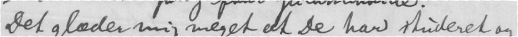Det glæder mig meget at De har studeret og
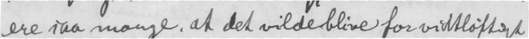ere saa mange, at det vilde blive for vidløftigt
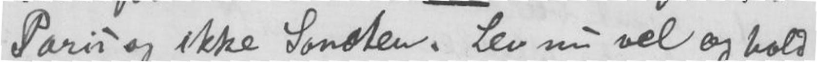Paris og ikke Sonaten. Lev nu vel og hold
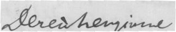Deres hengivne
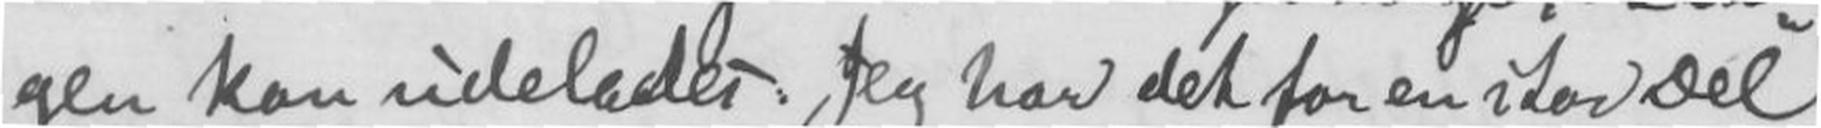gen kan udelades. Jeg har det for en stor Del
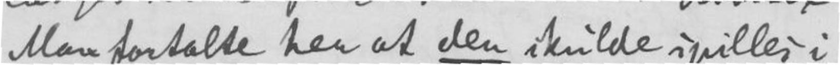Man fortalte her at den skulde spilles i
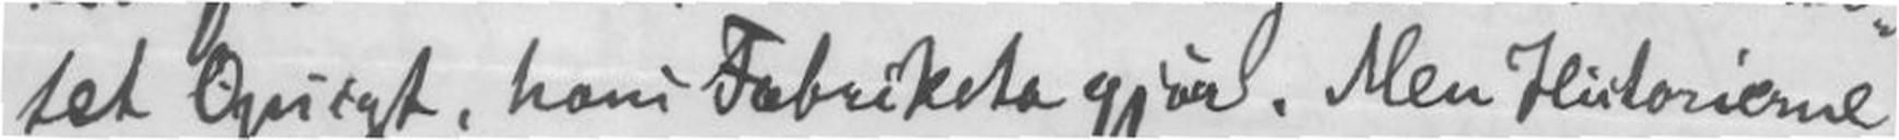tet Opsigt, hans Fabriketa gjør. Men Historierne
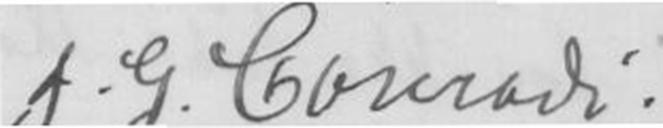J.G. Conradi
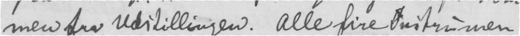men fra Udstillingen. Alle fire Instrumen-
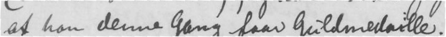at han denne Gang faar Guldmedaille.
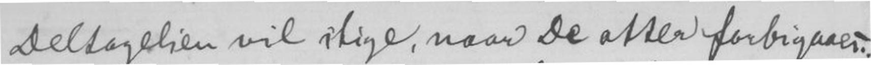Deltagelsen vil stige, naar De atter forbigaaes.
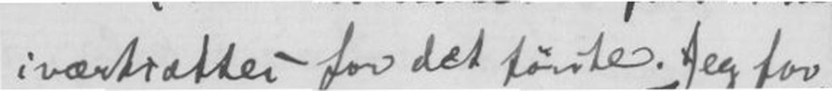iværtsættes for det første. Jeg for-
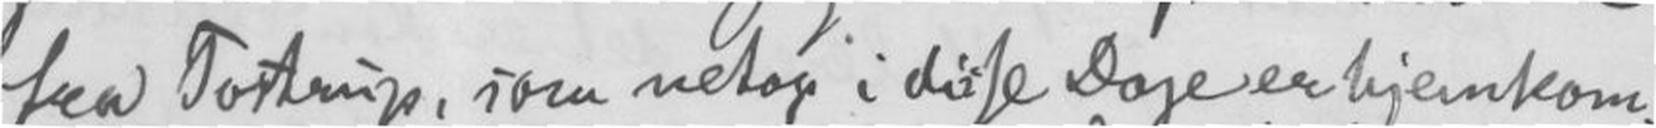fra Tostrup, som netop i disse Dage er hjemkom-
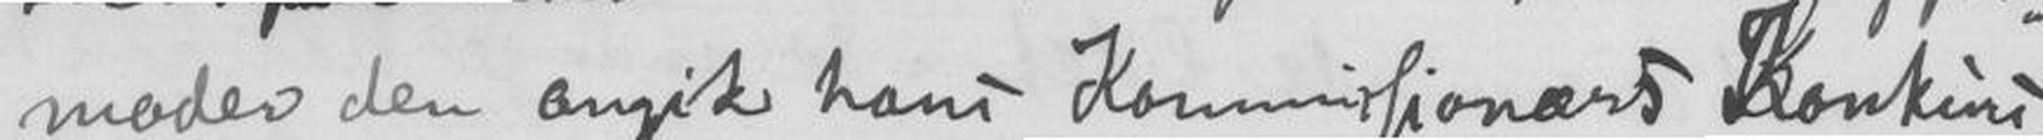moder den angik hans Kommissonærs Konkurs
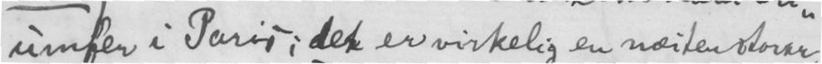umfer i Paris; det er virkelig en næsten storar
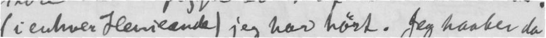(i enhver Henseænde) jeg har hørt. Jeg haaber da
